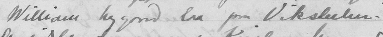William Nygaard laa paa Viksholm.
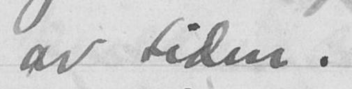av tiden.
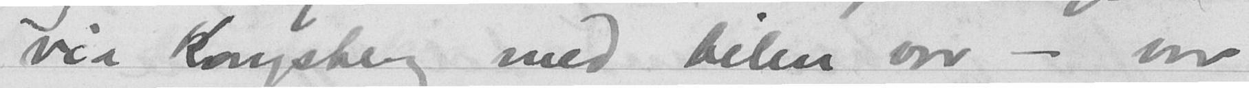via Kongsberg med bilen vaar - var
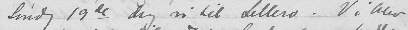Lørdag 19de drog vi til Lillero. Vi blev
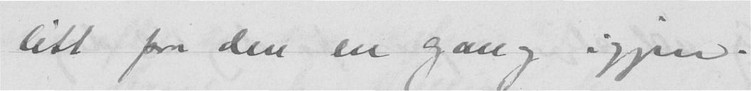litt paa den en gang igjen.
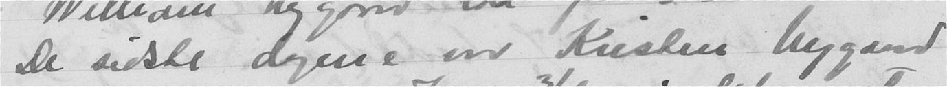De sidste dagene var Kristen Nygaard
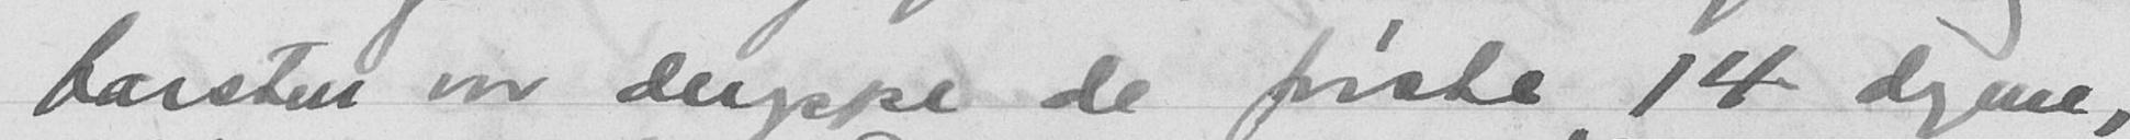Carsten var deroppe de første 14 dagene,
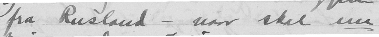fra Rusland - naar skal nu
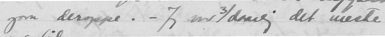ogsaa deroppe. - Jeg var 3/ daarlig det meste
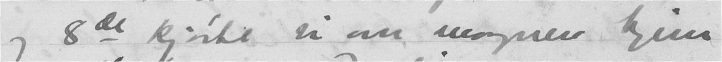og 8de kjørte vi om morgenen hjem
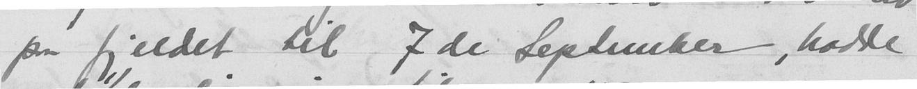paa fjeldet til 7de September, hadde
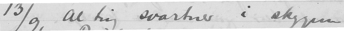13/9 Al ting svartner i skygen
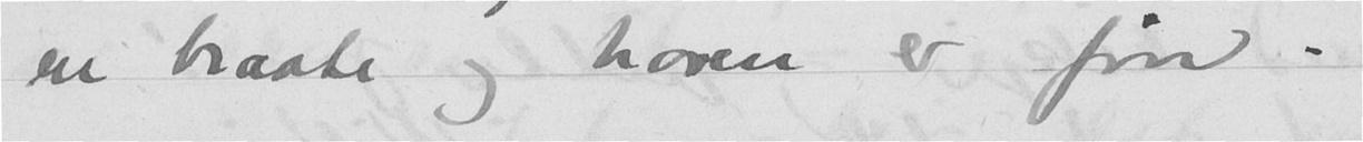en braate og haven er fin.
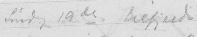Lørdag 19de. Liefjeld
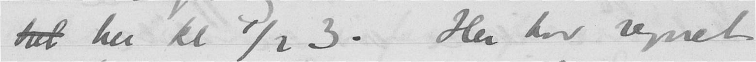til her kl ½ 3. Her har regnet
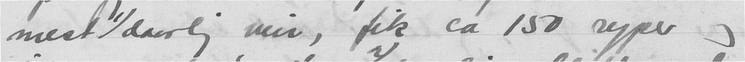mest daarlig veir, fik ca 150 ryper og
